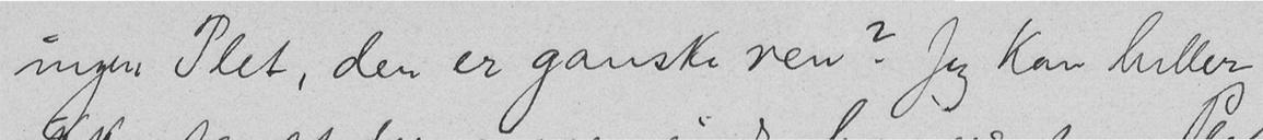ingen Plet, den er ganske ren? Jeg kan heller
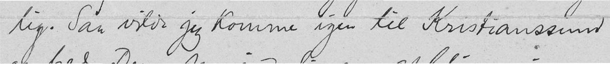lig. Saa vilde jeg komme igen til Kristianssund
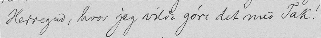Herregud, hvor jeg vilde gøre det med Tak!
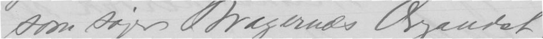som søger Bragernæs Organist-
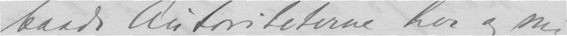baade Autoriteterne her og mig
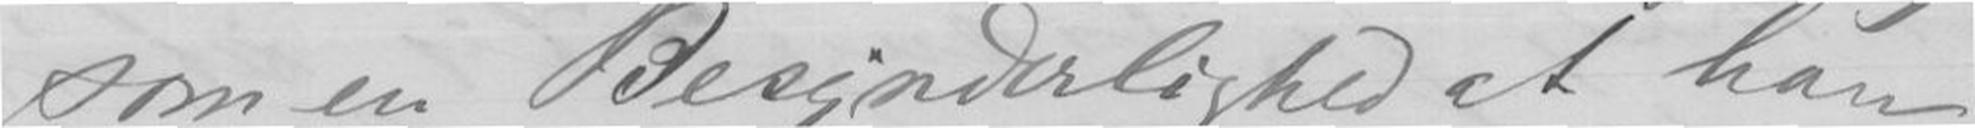som en Besynderlighed at han
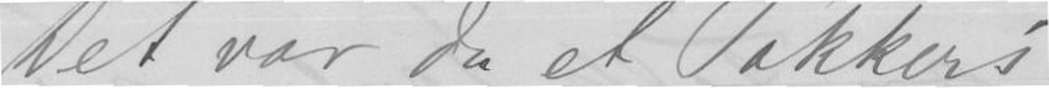Det var da et Pokkers
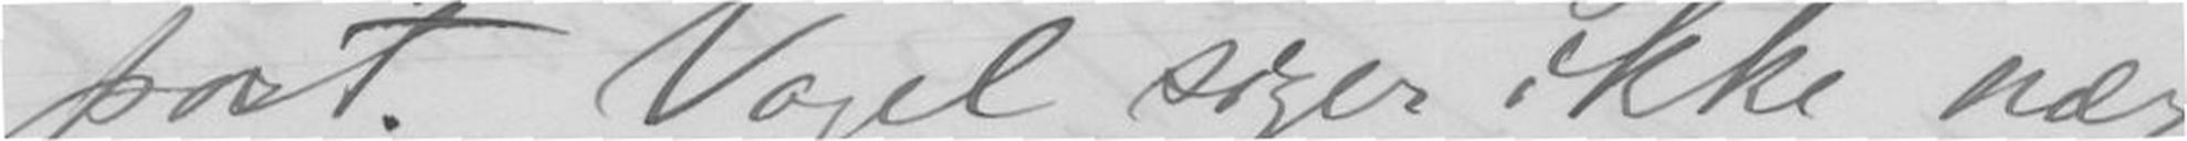post. Vojel siger ikke nær
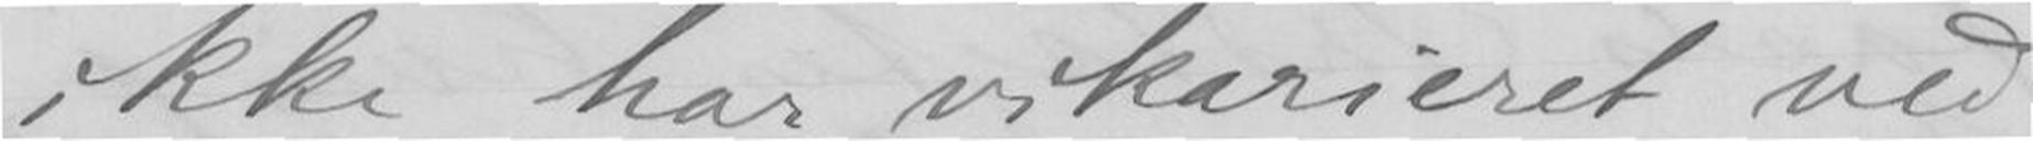ikke har vikarieret ved
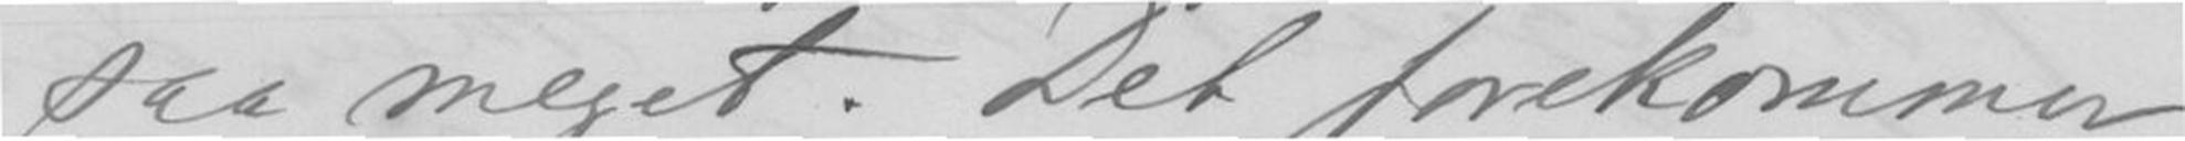saa meget. Det forekommer
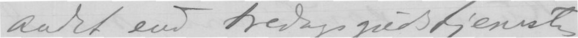andet end Fredagsgudstjenester
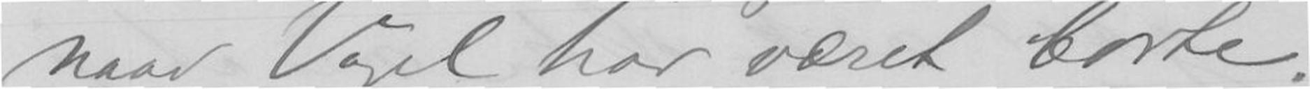naar Vojel har været borte.
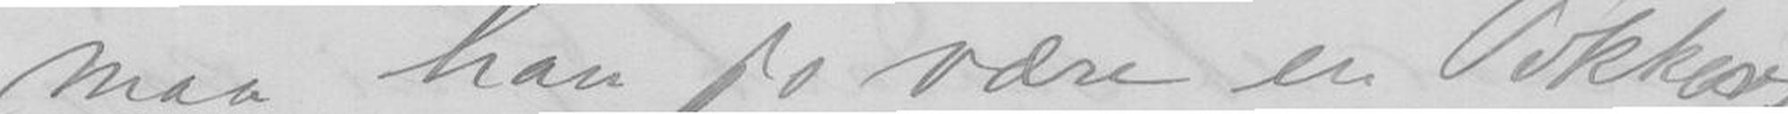maa han jo være en Pokkers
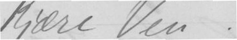Kjære Ven!
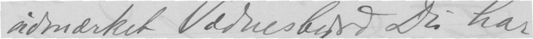udmærket Vidnesbyrd Du har
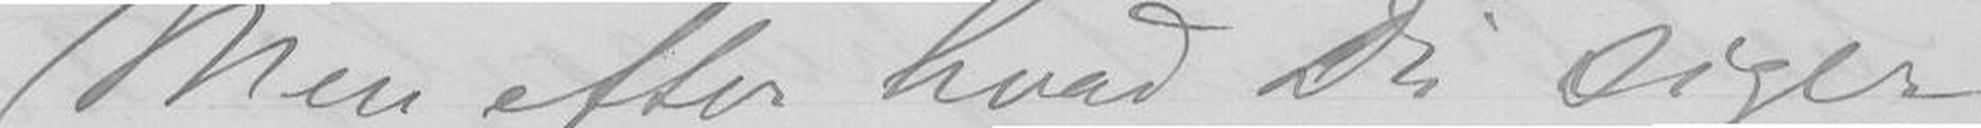Men efter hvad Du siger
